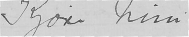Kjære Nini,
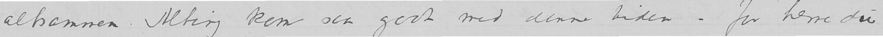altsammen. Alting kom saa godt med denne tiden – for herre du
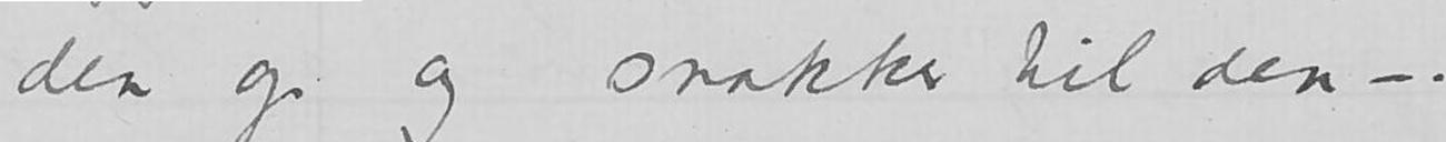den op og snakker til den –.
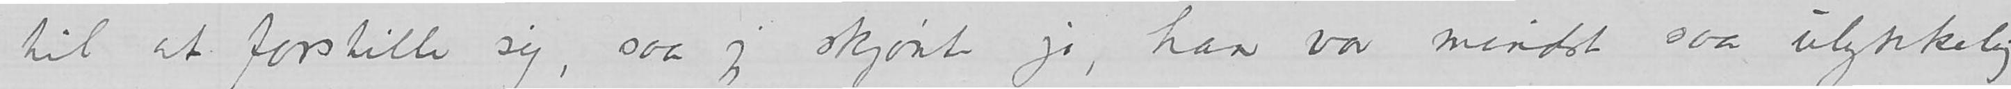til at forstille sig, saa jeg skjønte jo, han var mindst saa ulykkelig
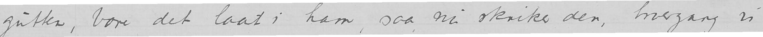gutten, bare det laat i ham, saa nu skriker den, hvergang vi
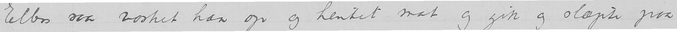Ellers saa vasket han op og hentet mat og gik og slæpte paa
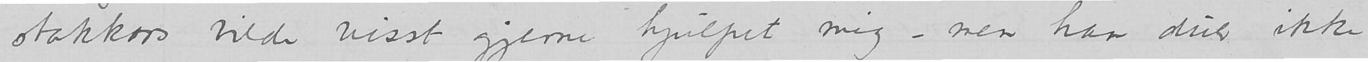stakkars vilde visst gjerne hjulpet mig – men han duer ikke
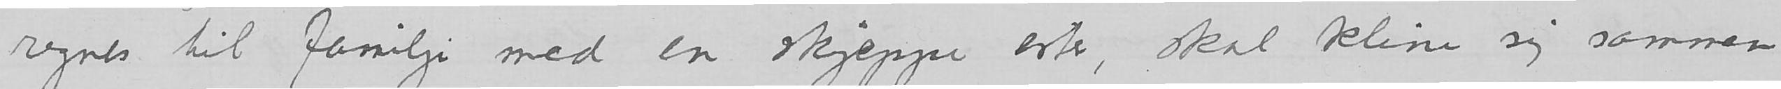synes til familje med en skjeppe erter, skal kline sig sammen
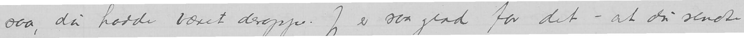saa, du hadde været deroppe. Jeg er saa glad for det – at du sendte
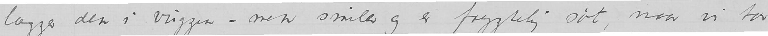lægger den i vuggen – men smiler og er frygtelig søt, naar vi tar
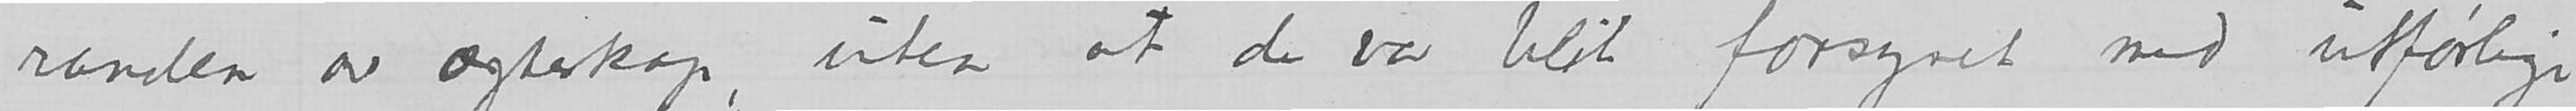randen av ægteskap, uten at de var blit forsynet med udførlige
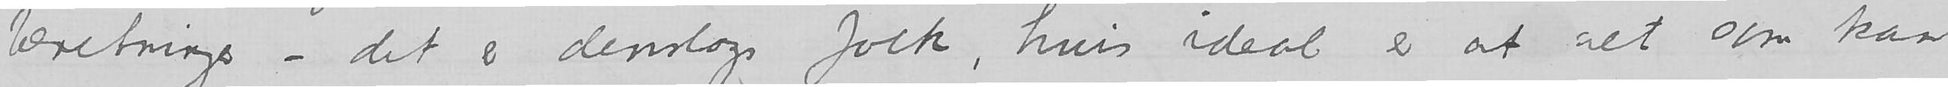beretninger – det er denslags folk, hvis ideal er at alt som kan
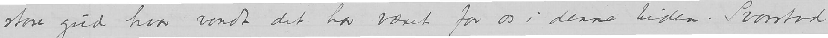store gud hva vondt det har været for os i denne tiden. Svarstad
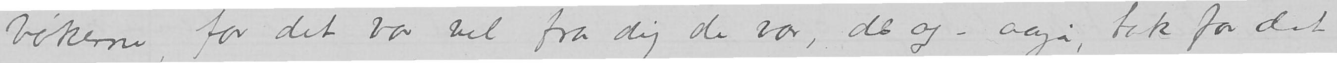bøkerne, for det var vel fra dig de var, de og – aaja, tak for det
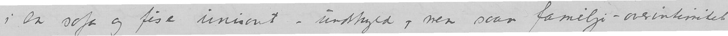i en sofa og fise unisont – undskyld, men saan familje-overintimitet
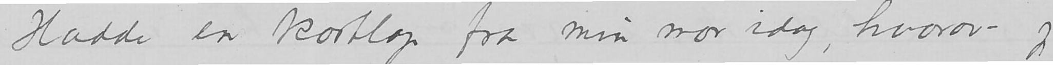Hadde en kortlap fra min mor idag, hvorav jeg
